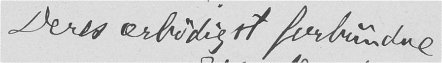Deres ærbødigst forbundne
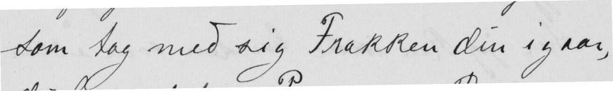som tog med sig Frakken din igaar,
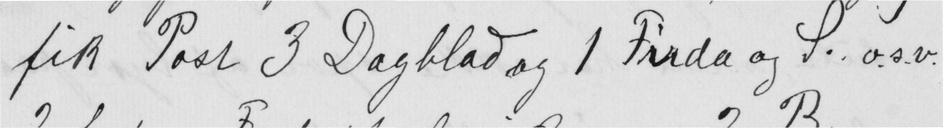fik Post 3 Dagblad og I Firda og P. o.s.v.
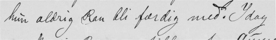hun aldrig kan bli færdig med. Idag
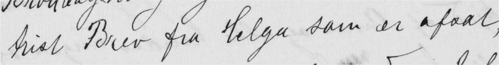hist Brev fra Velga som er afsat,
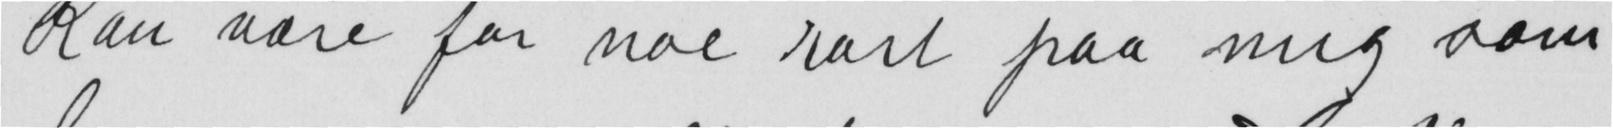kan vare for noe kart paa mig som
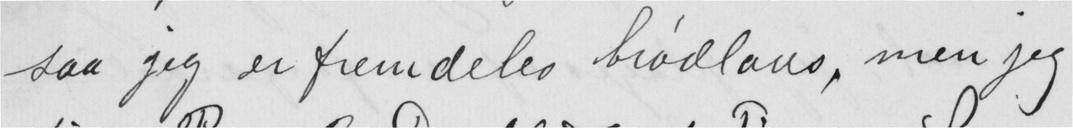saa jeg er fremdeles brødlaus men jeg
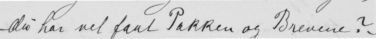du har vel faat Pakken og Brevene?
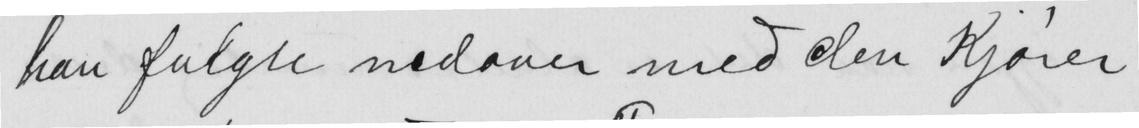han fulgte nedover med den Kjører
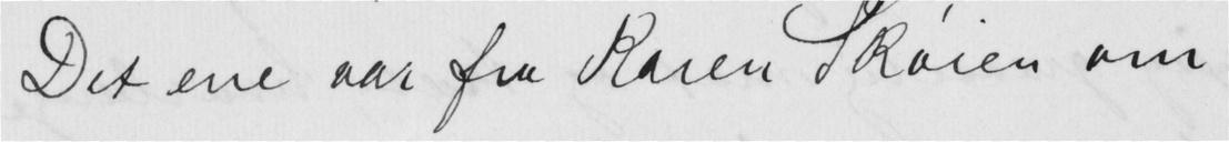Det ene aar fra Karen Køien om
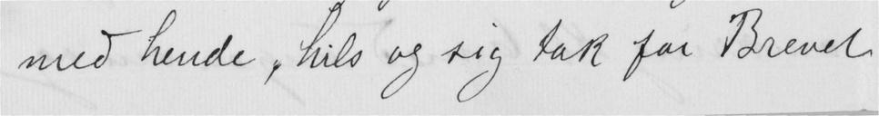med hende, hils og sig tak for Brevet
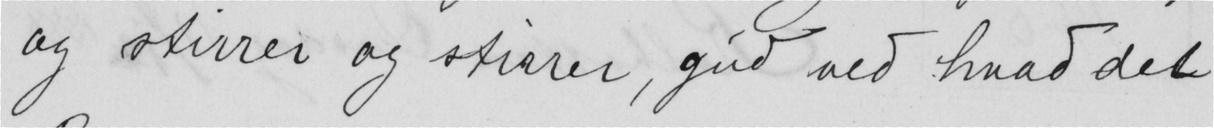og stirrer og stirrer, gud ved hvad det
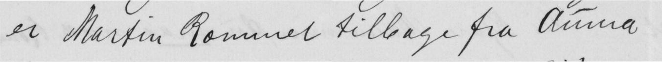er Martin Rommel tilbage fra Amma
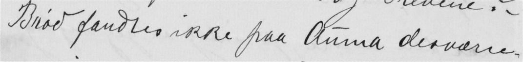Brød fandtes ikke paa Auma desværre
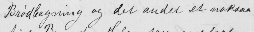Brødbagning og det andet er noksaa
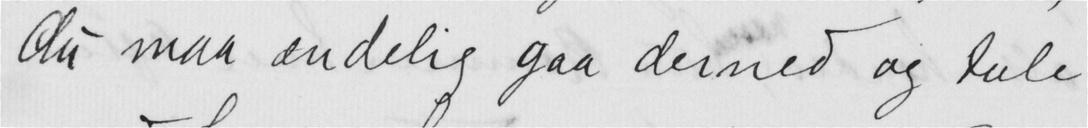Du maa endelig gaa derned og tule
